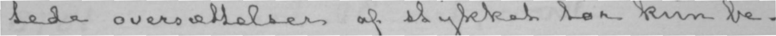tede oversættelser af stykket tør kun be-
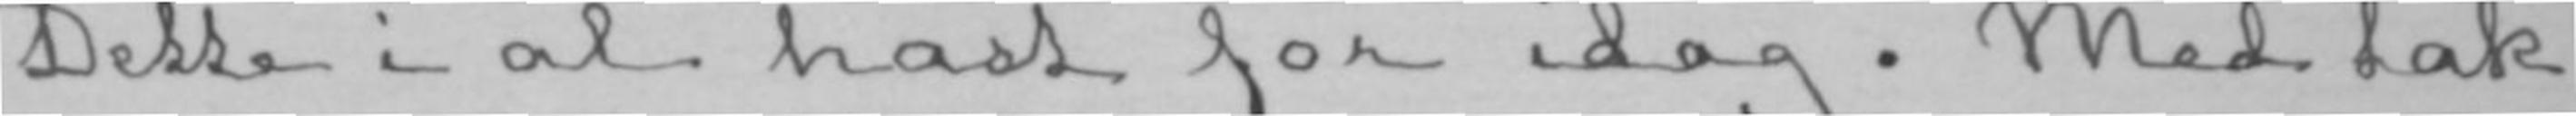Dette i al hast for idag. Med tak
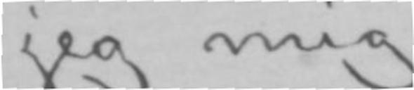jeg mig
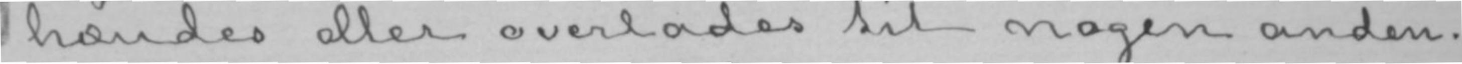hændes eller overlades til nogen anden.
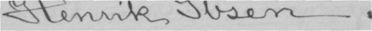Henrik Ibsen.
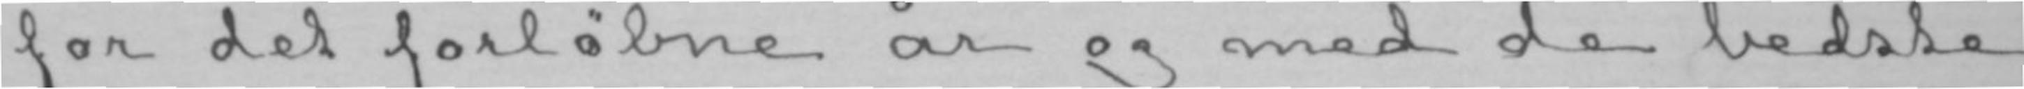for det forløbne år og med de bedste
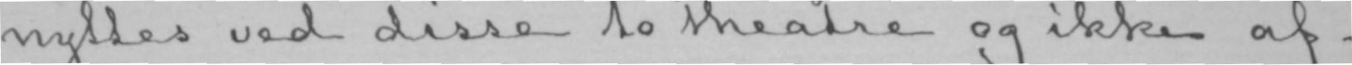nyttes ved disse to theatre og ikke af-
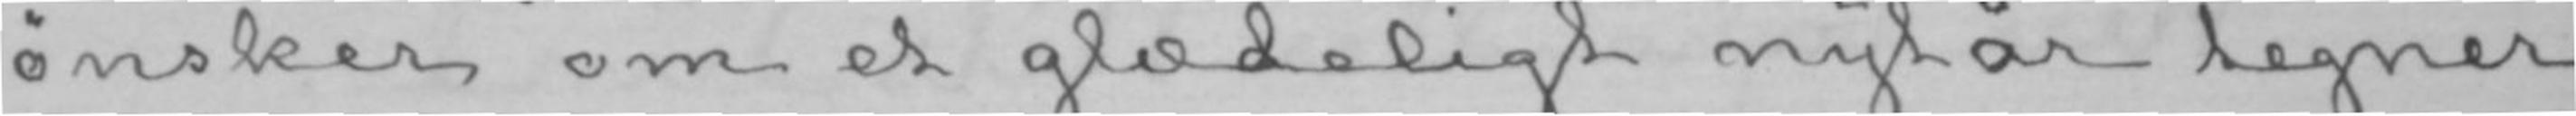ønsker om et glædeligt nytår tegner
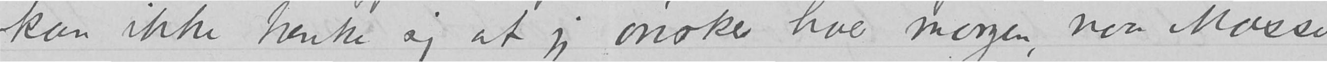kan ikke tænke sig at jeg ønsker hver morgen, naar Mosse
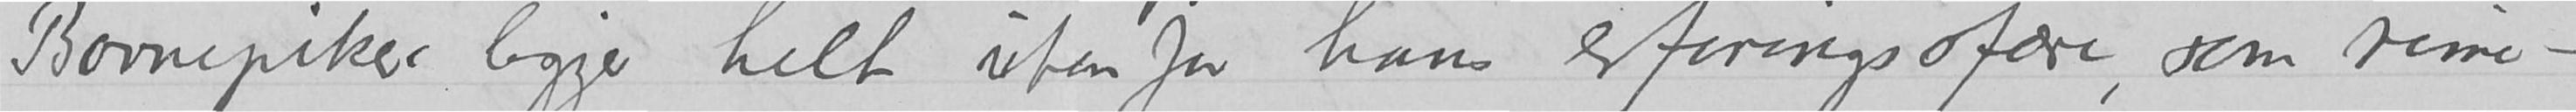Barnepiker ligger helt utenfor hans erfaringssfære, som rime-
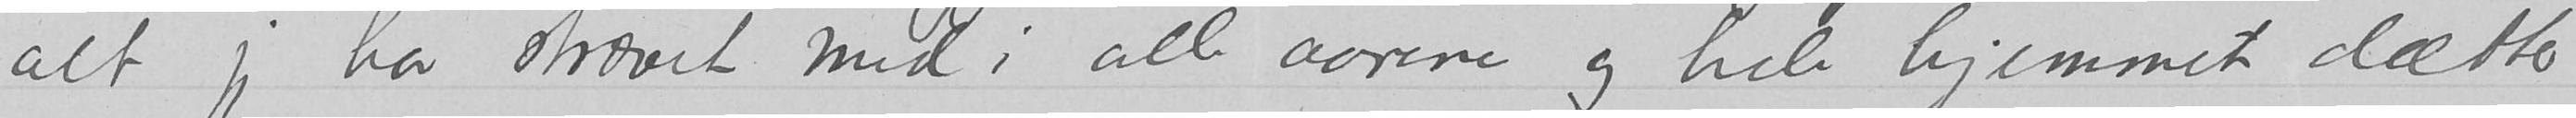alt jeg har strævet med i alle aarene og hele hjemmet dætter
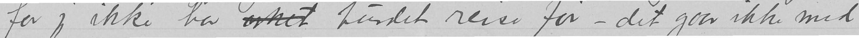for jeg ikke har orket turdet reise før – det gaar ikke med
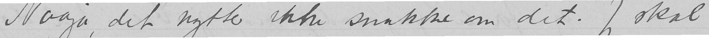Naaja, det nytter ikke snakke om det. Jeg skal
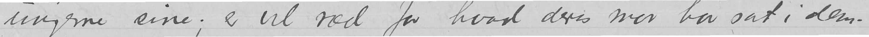ungerne sine; er vel ræd for hvad deres mor har sat i dem.
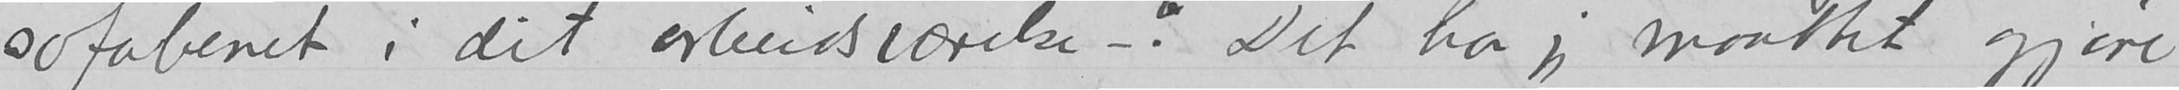sofabenet i dit arbeidsværelse -? Det har jeg maadtet gjøre
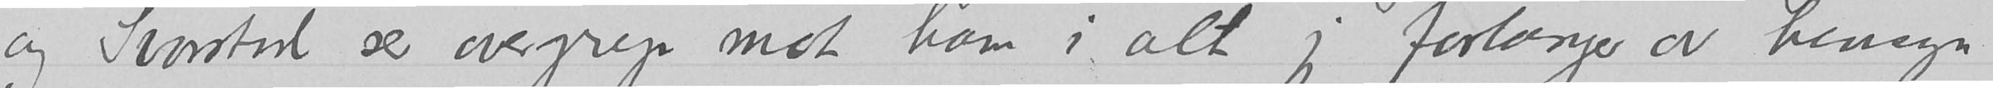og Svarstad ser overgrep mot ham i alt jeg forlanger av hensyn
